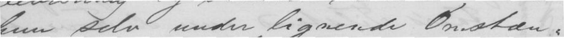hun selv under lignende Omstæn-
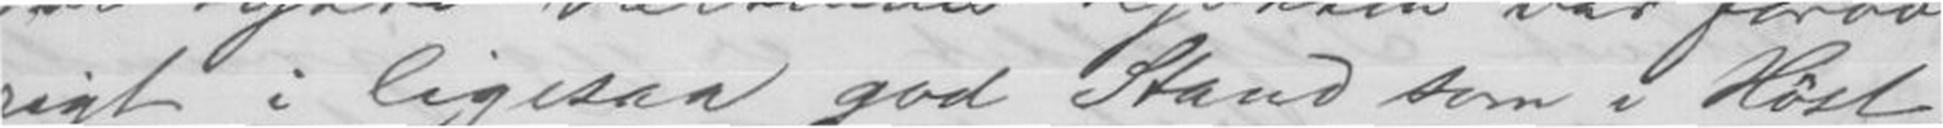rigt i ligesaa god Stand som i Høst,
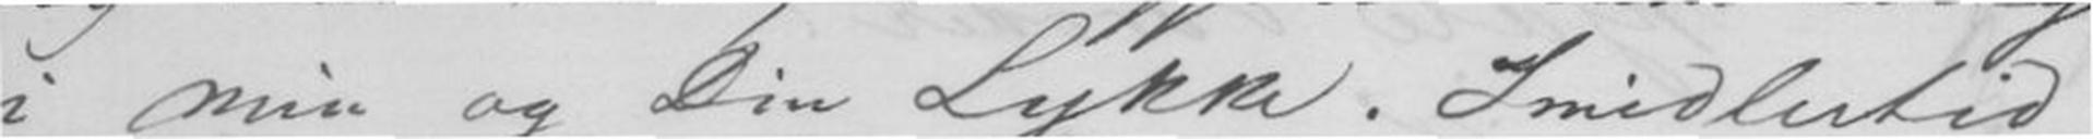i min og din Lykke. Imidlertid
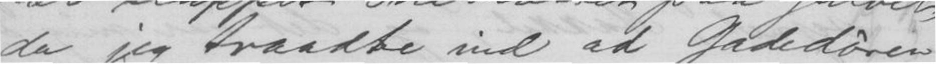da jeg traadte ind ad Gadedøren
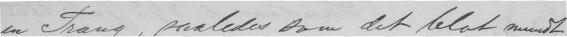en Trang, saaledes som det blot mundt-
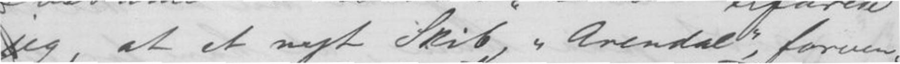jeg, at et nyt Skib, Arendal, forven-
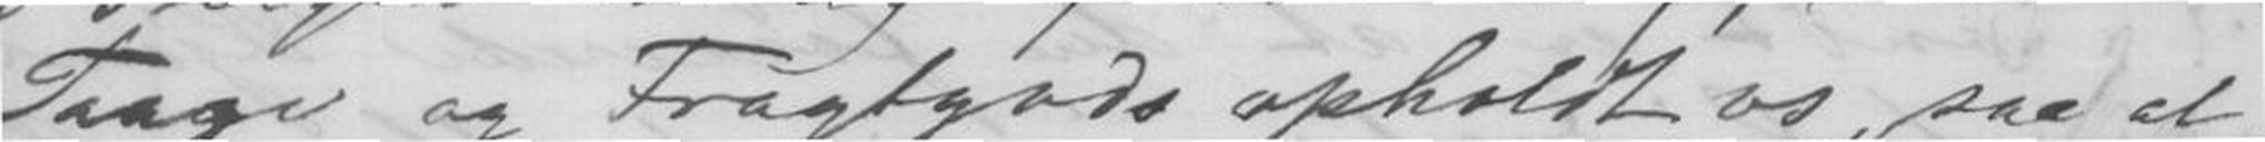Taage og Fragtgods opholdt os, saa at
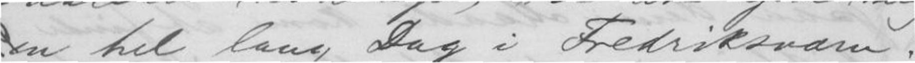en hel lang Dag i Fredriksværn.
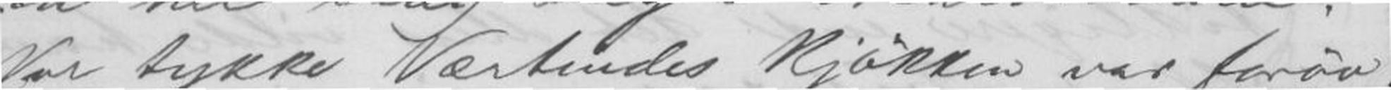Vor tykke Værtindes Kjøkken var forøv-
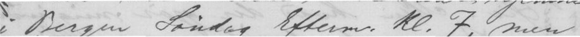i Bergen Søndag Efterm. Kl.7, men
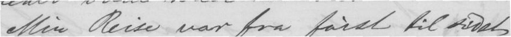Min Reise var fra først til sidst
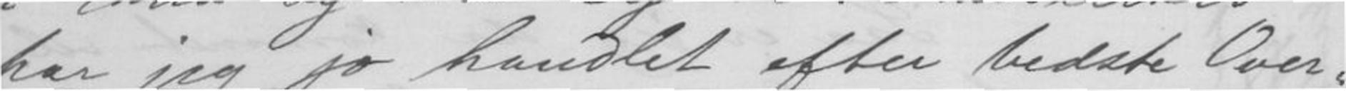har jeg jo handlet efter bedste Over-
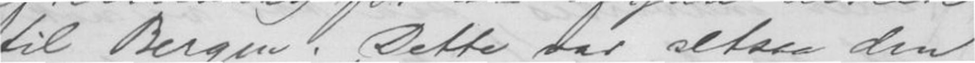til Bergen. Dette var altsaa den
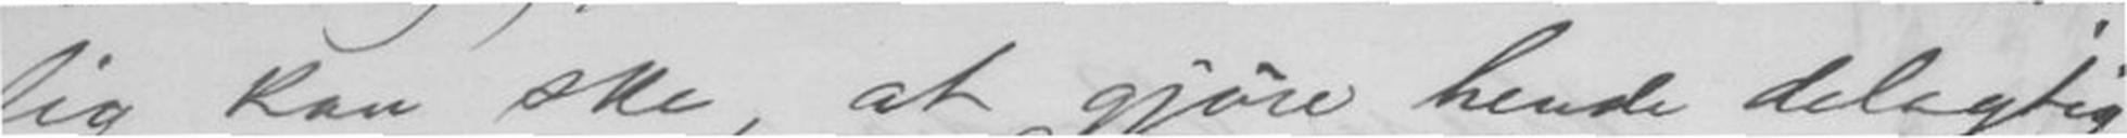lig kan ske, at gjøre hende delagtig
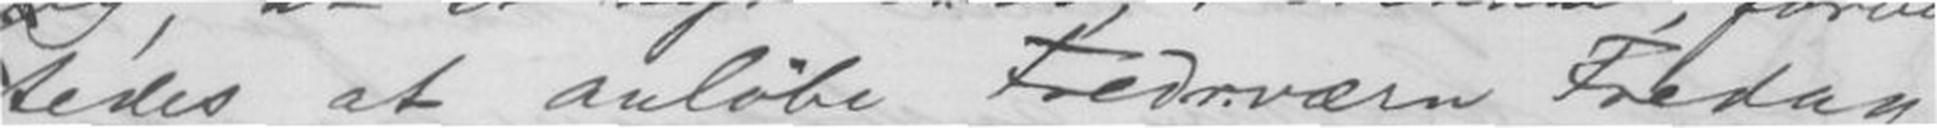tedes at anløbe Fredr.værn Fredag
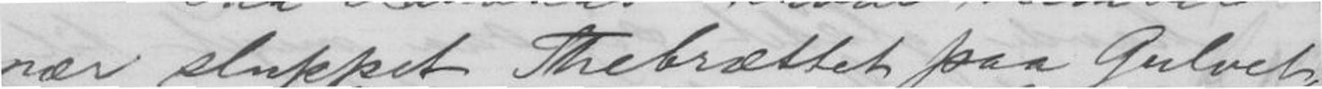nær sluppet Thebrættet paa Gulvet,
